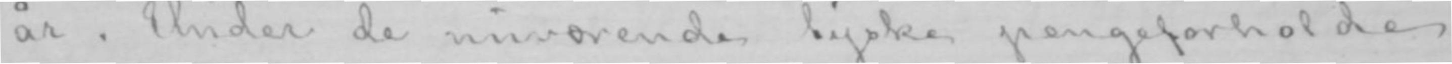år. Under de nuværende tyske pengeforholde
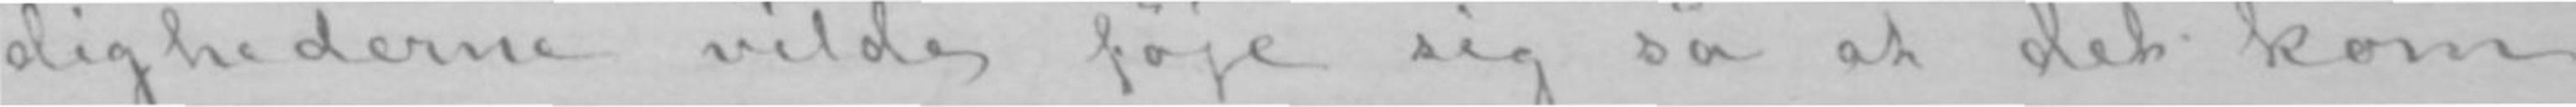dighederne vilde føje sig så at det kom
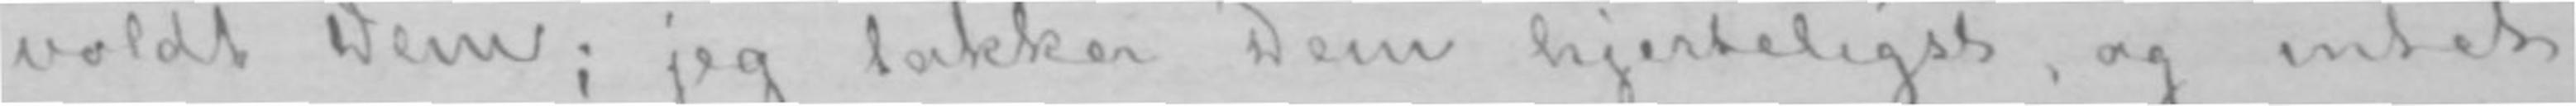voldt Dem; jeg takker Dem hjerteligst, og intet
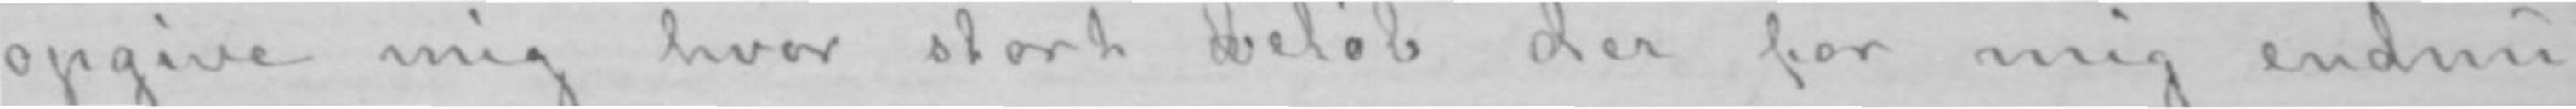opgive mig hvor stort beløb der for mig endnu
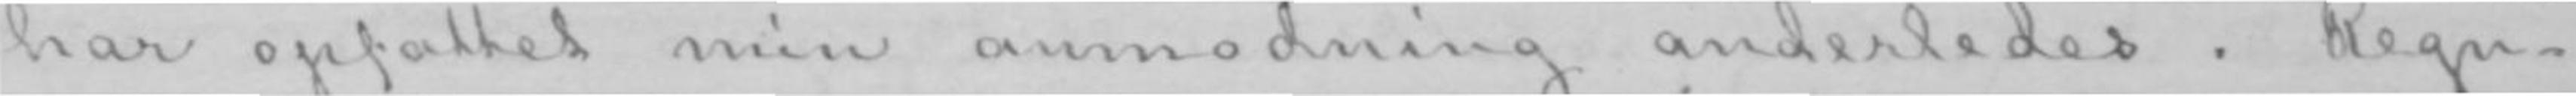har opfattet min anmodning anderledes. Regn-
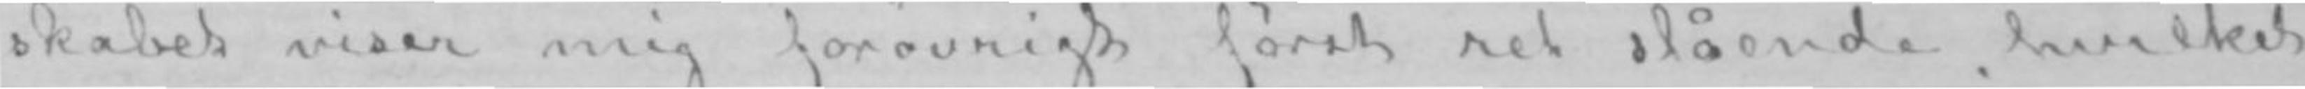skabet viser mig forøvrigt først ret slående, hvilket
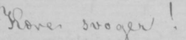Kære svoger!
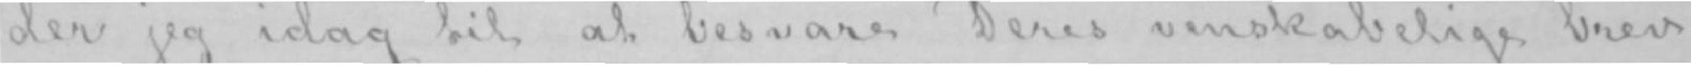der jeg idag til at besvare Deres venskabelige brev
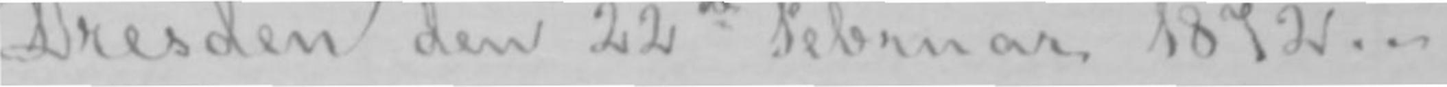Dresden den 22de Februar 1872.-
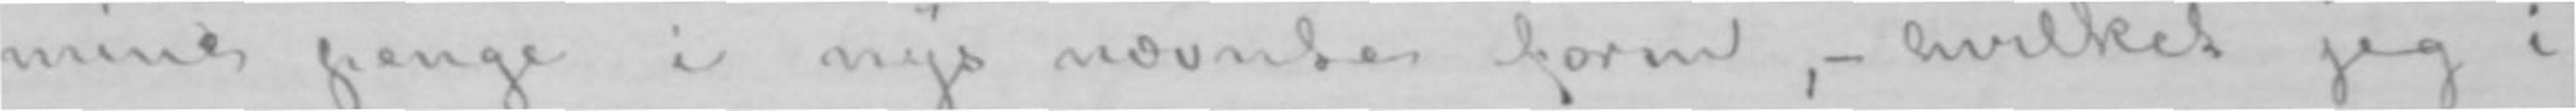mine penge i nys nævnte form,- hvilket jeg i
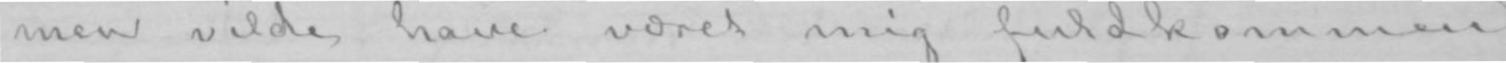men vilde have været mig fuldkommen
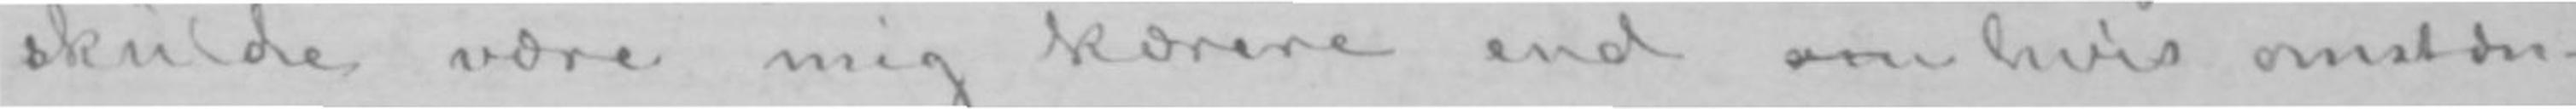skulde være mig kærere end om hvis omstæn-
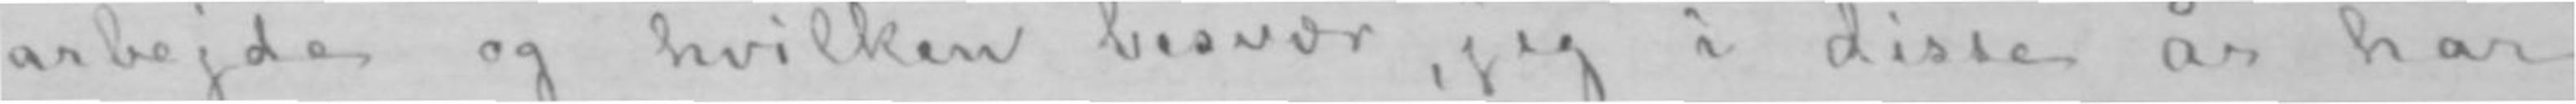arbejde og hvilken besvær, jeg i disse år har
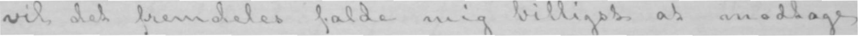vil det fremdeles falde mig billigst at modtage
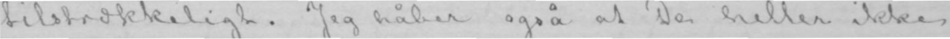tilstrækkeligt. Jeg håber også at De heller ikke
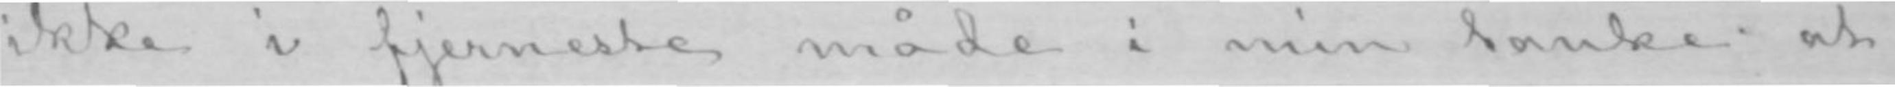ikke i fjerneste måde i min tanke at
